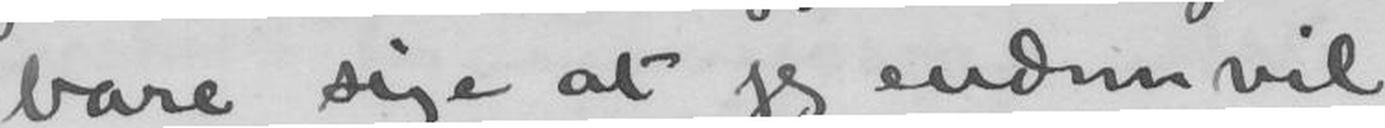bare sige at jeg endnu vil
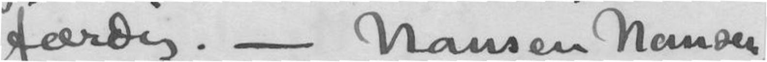færdig. -- Nansen, Nansen,
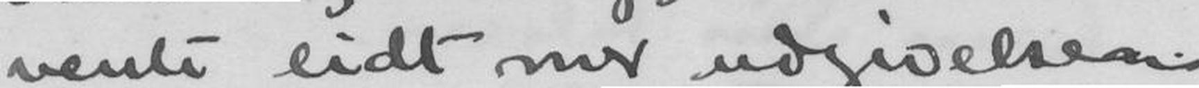vente lidt med udgivelsen.
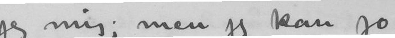jeg mig; men jeg kan jo
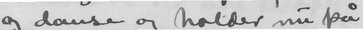og danse og holder nu på
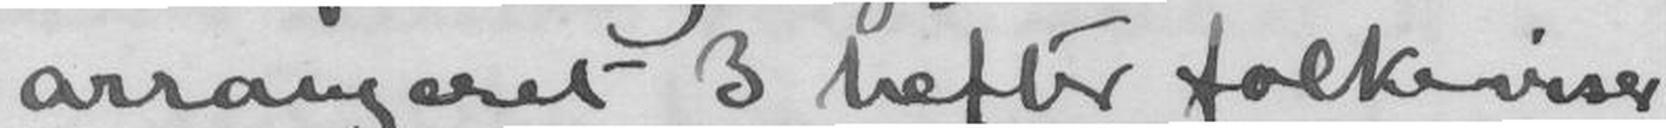arrangeret 3 hefter folkeviser
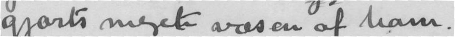gjorts meget væsen af ham.
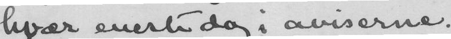hvær eneste dag i aviserne.
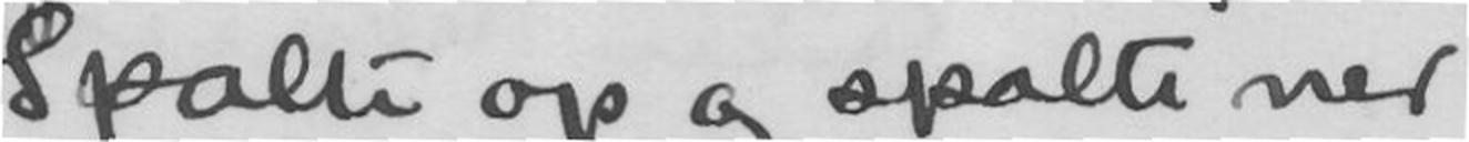Spalte op og spalte ned
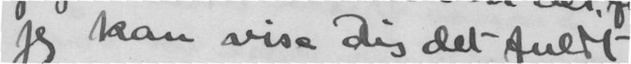jeg kan vise Dig det fuldt
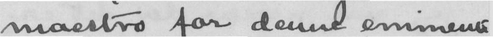maestro for denne eminente
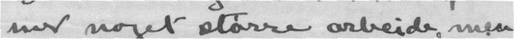med noget større arbeide, men
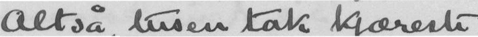Altså, tusen tak kjæreste
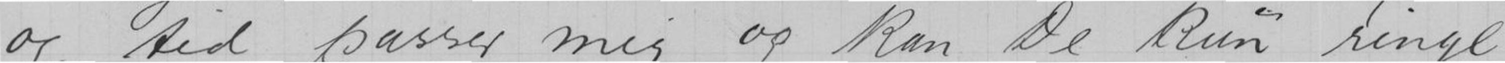og tid passer mig og kan De kun ringe
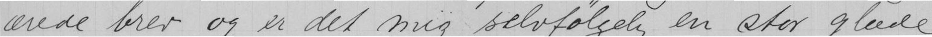ærede brev og er det mig selvfølgelig en stor glæde
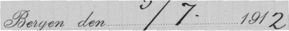Bergen den 5/7 1912
